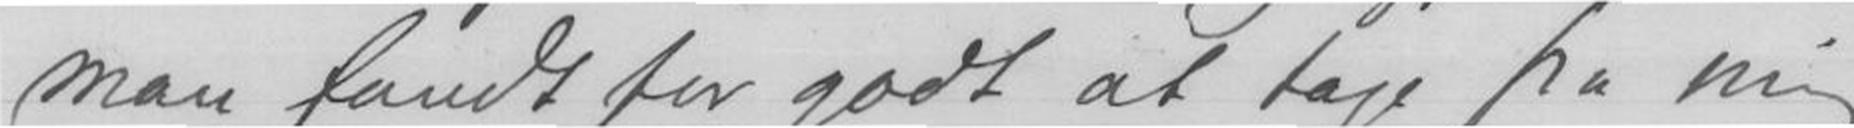man fandt for godt at tage fra mig
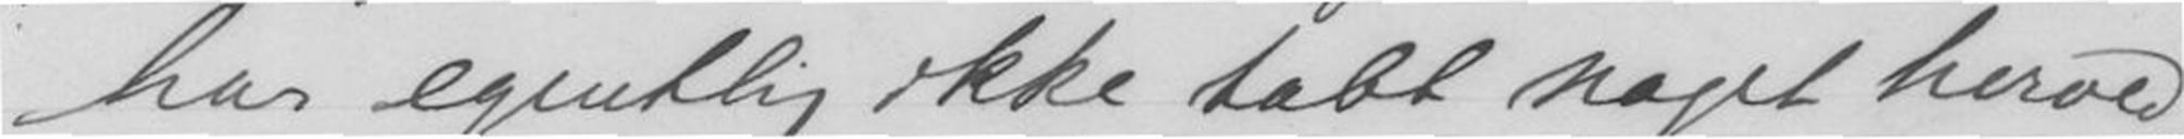har egentlig ikke tabt noget herved,
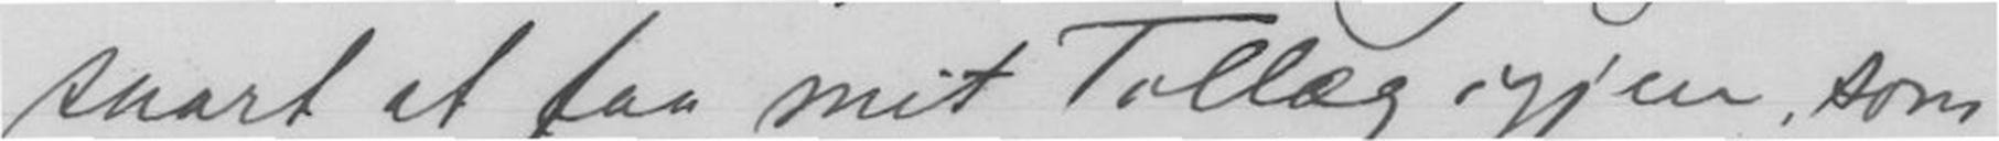snart at faa mit Tillæg igjen som
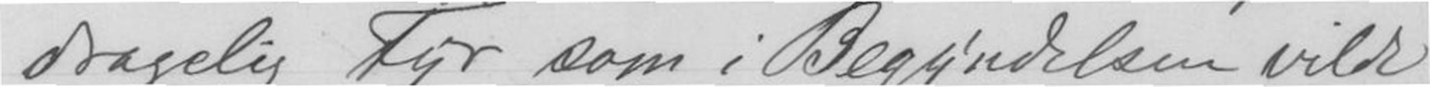dragelig Fyr som i Begyndelsen vilde
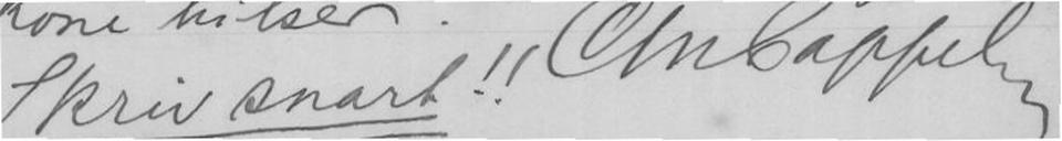Skriv snart!! Chr. Cappelen
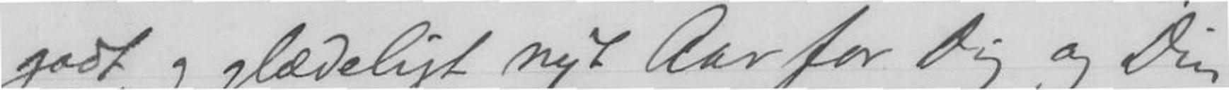godt og glædeligt nyt Aar for Dig og Din
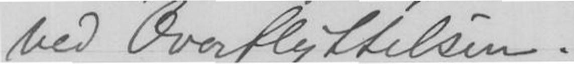ved Overflyttelsen.
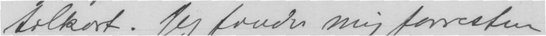tilkort. Jeg finder mig forresten
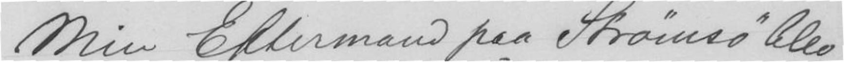Min Eftermand paa Strømsø blev
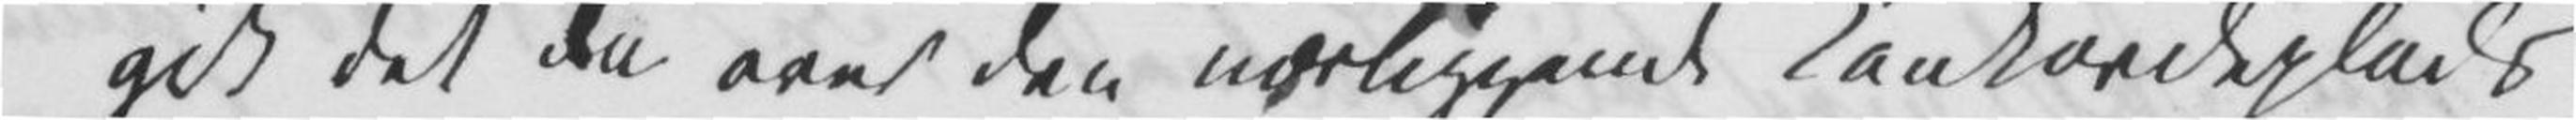gik det da over den nærliggende Konkordeplads
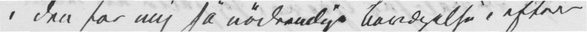i den for mig så nødvendige Bevægelse, efter¬
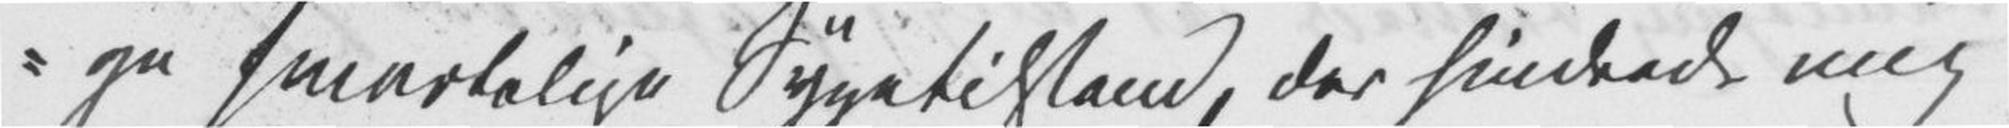ge smertelige Sygetilstand, der hindrede mig
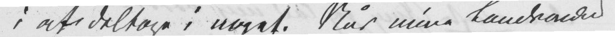i at deltage i noget. Når mine Landsmænd¬
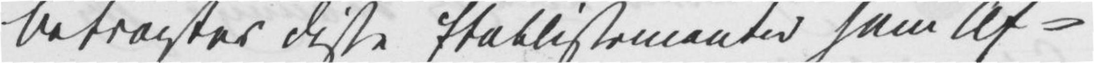betragter disse Etablissementer som Af¬
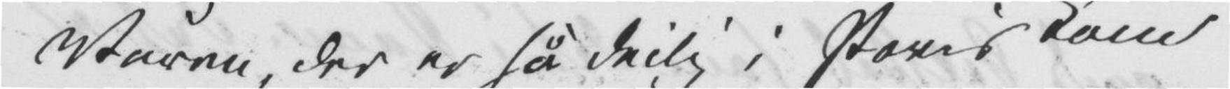Våren, der er så deilig i Paris kom
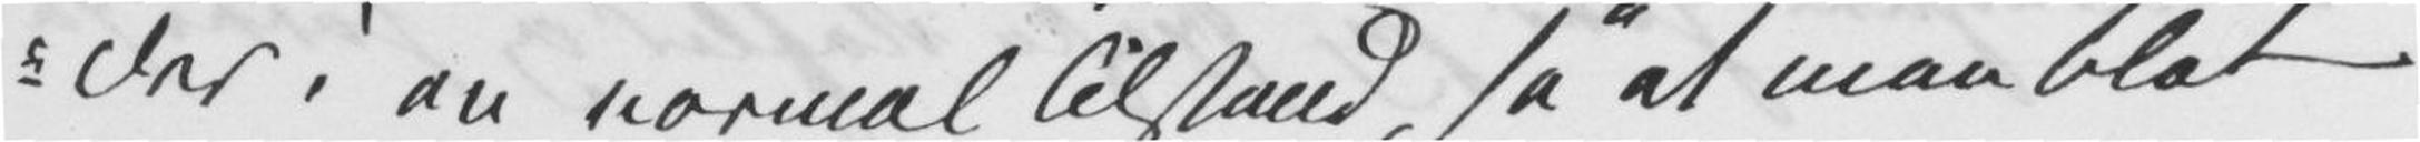der i en normal Tilstand, så at man blot
